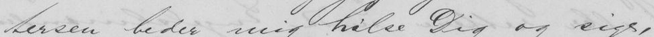tersen beder mig hilse Dig og siger,
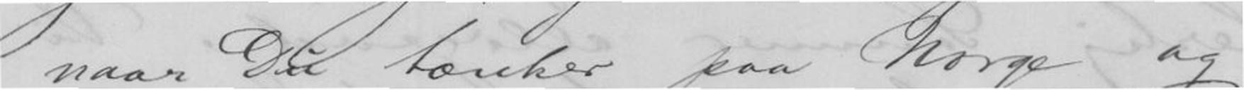naar Du tænker paa Norge og
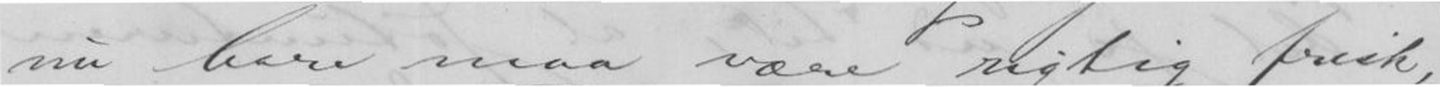nu bare maa være rigtig frisk,
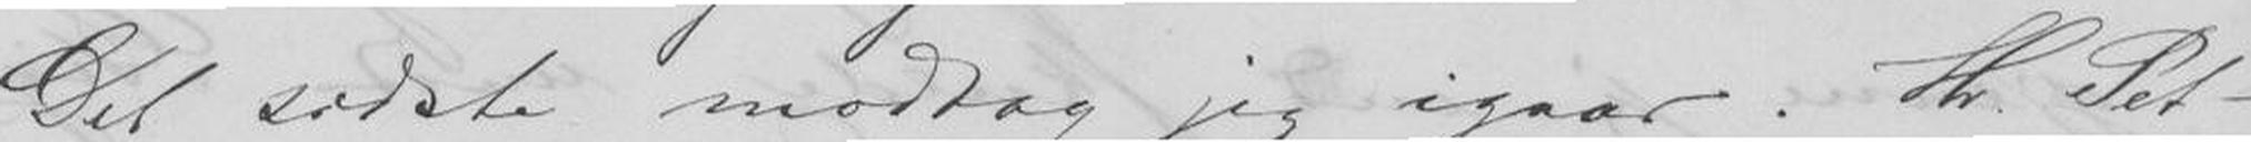Det sidste modtog jeg igaar. Hr. Pet-
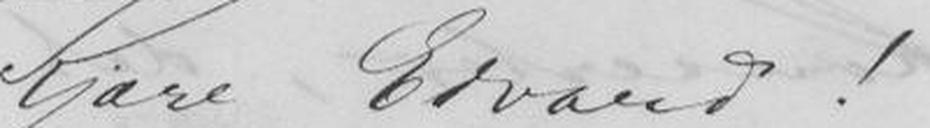Kjære Edvard!
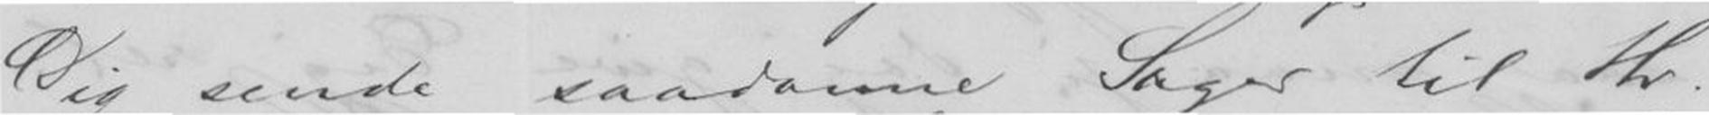Dig sende saadanne Sager til Hr.
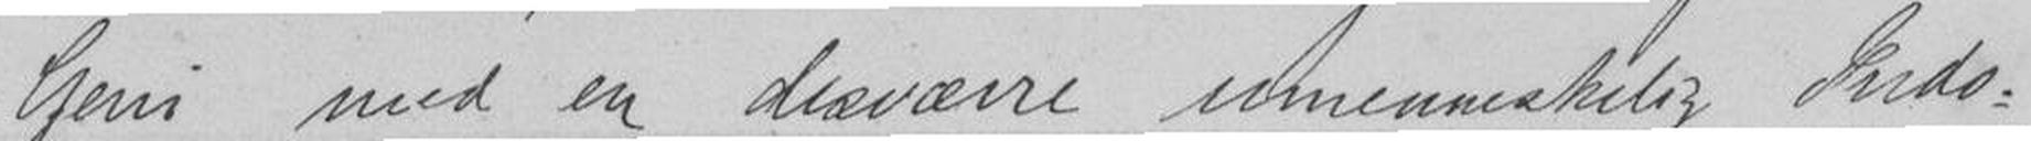Geni med en desværre umenneskelig Indo-
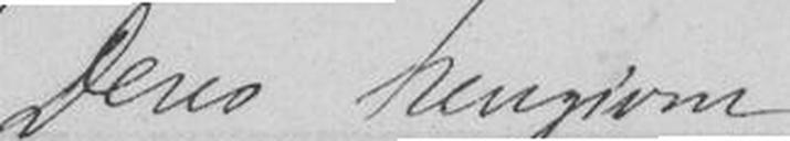Deres hengivne
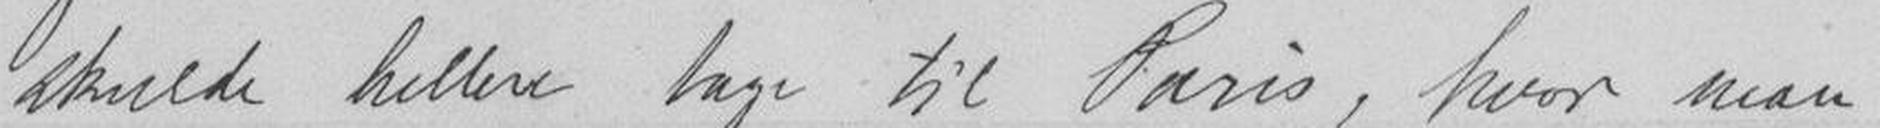skulde hellere tage til Paris, hvor man
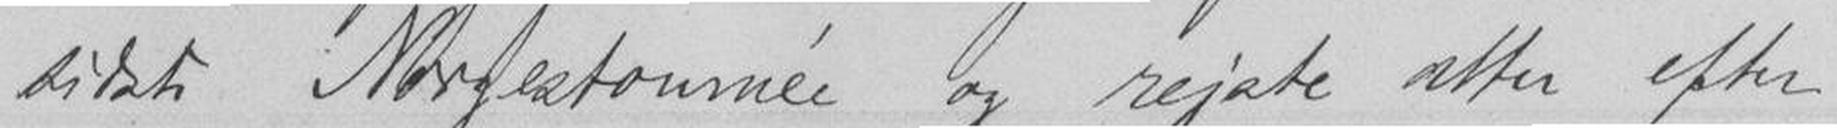sidste Norgestournée og rejste atter efter
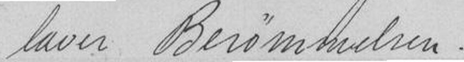laver Berømmelsen.
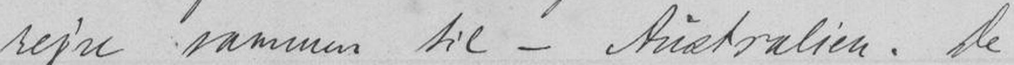rejse sammen til - Australien. De
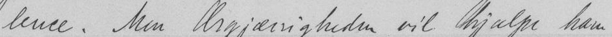lence, Men Ærgjærigheden vil hjælpe ham.
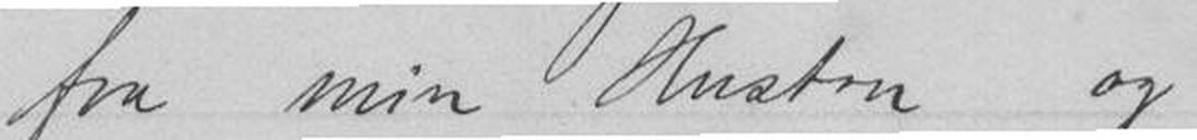fra min Hustru og
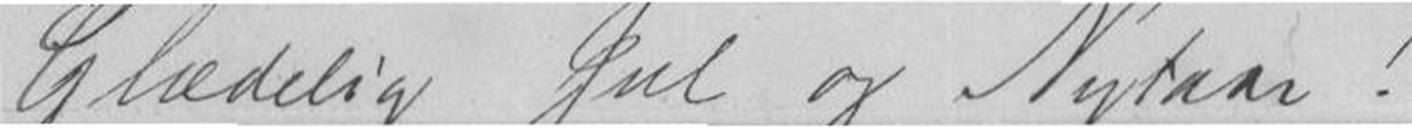Glædelig Jul og Nytaar!
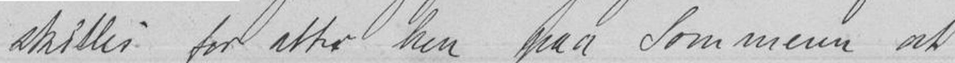skilles for atter hen paa Sommeren at
